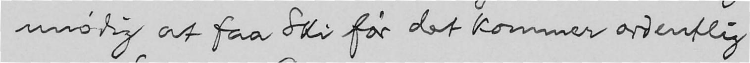unødig at faa Ski før det kommer ordentlig
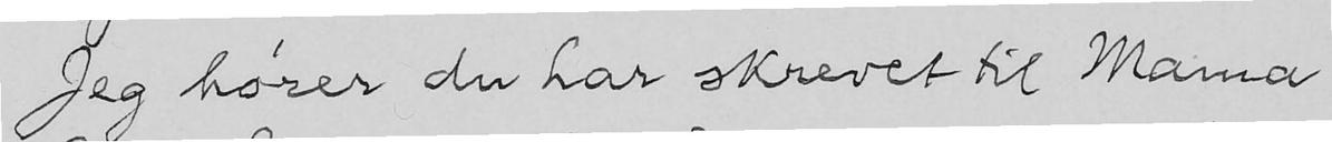Jeg hører du har skrevet til Mama
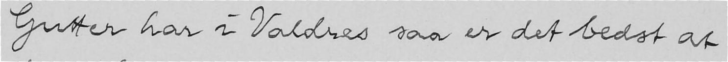Gutter har i Valdres saa er det bedst at
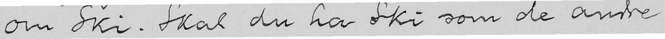om Ski. Skal du ha Ski som de andre
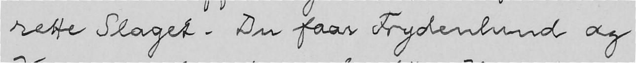rette Slaget. Du faar Frydenlund og
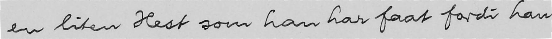en liten Hest som han har faat fordi han
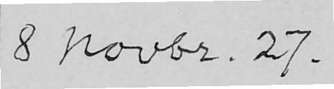8 Novbr. 27.
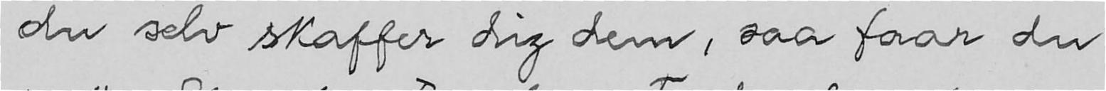du selv skaffer dig dem, saa faar du
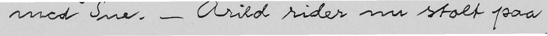med Sne. - Arild rider nu stolt paa
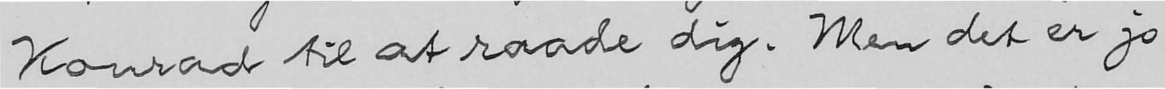Konrad til at raade dig. Men det er jo
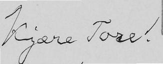Kjære Tore!
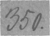350.
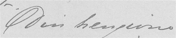Din hengivne
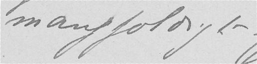mangfoldigt.
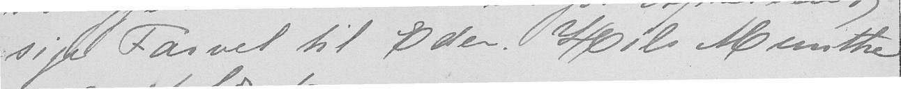sige Farvel til Eder. Hils Munthe
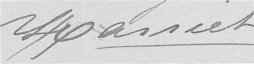Harriet
